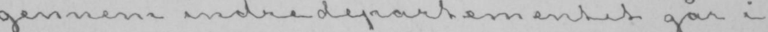gennem indredepartementet går i
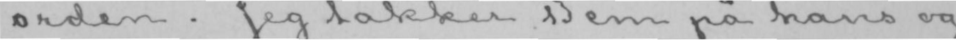orden. Jeg takker Dem på hans og
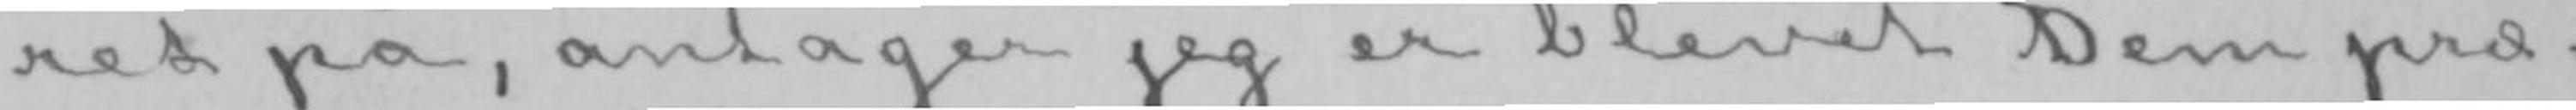ret på, antager jeg er blevet Dem præ-
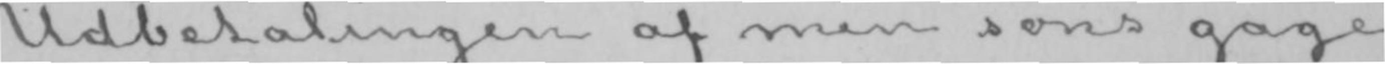Udbetalingen af min søns gage
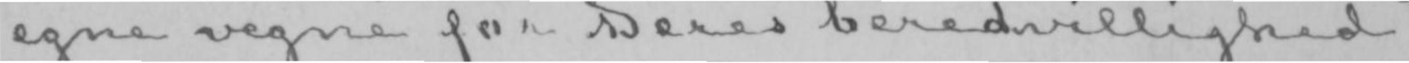egne vegne for Deres beredvillighed
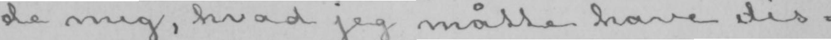de mig, hvad jeg måtte have dis-
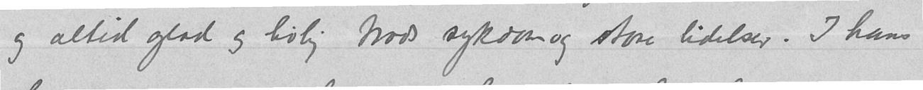og altid glad og livlig trods sykdom og store lidelser. I hans
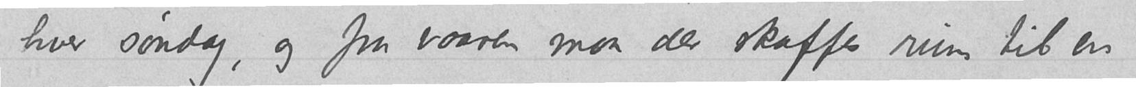hver søndag, og fra vaaren maa der skaffes rum til en
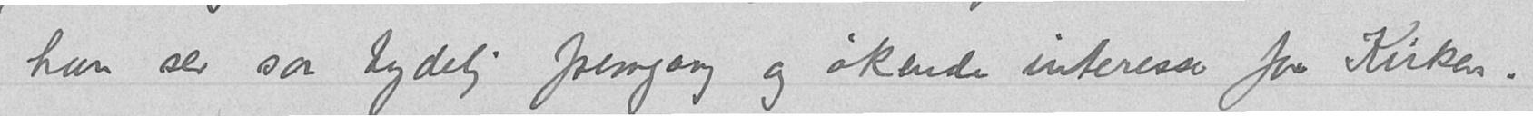han ser saa tydelig fremgang og økende interesse for Kirken.
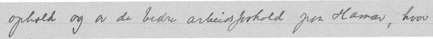ophold og av de bedre arbeidsforhold paa Hamar, hvor
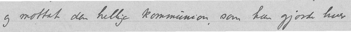og mottat den hellige kommunion, som han gjorde hver
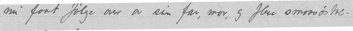nu faat følge over av sin far, mor, og flere smaasøstre.
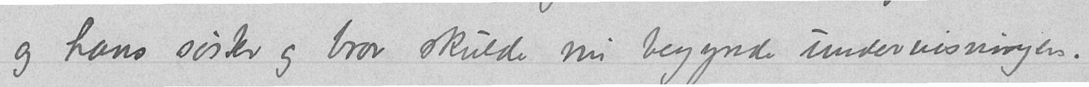og hans søster og bror skulde nu begynde undervisningen.
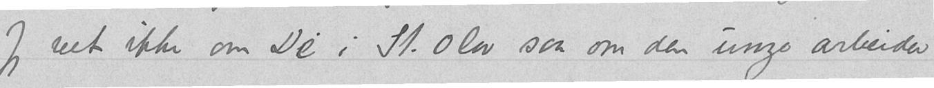Jeg vet ikke om De i St. Olav saa om den unge arbeider
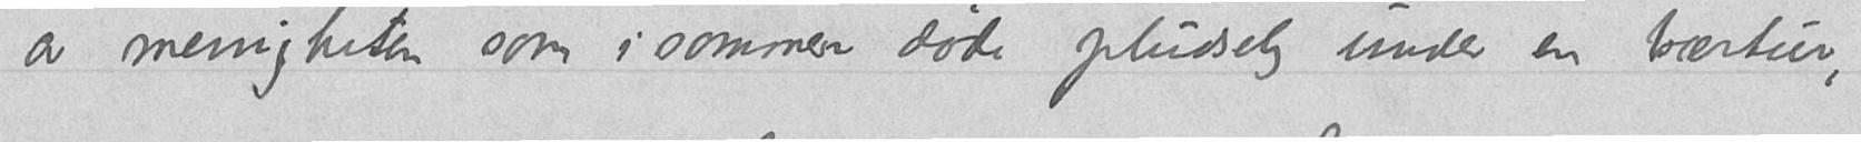av menigheten som i sommer døde pludselig under en bærtur,
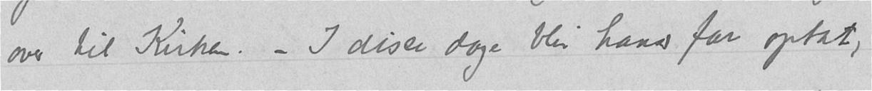over til Kirken. – I disse dager blir hans far optat,
